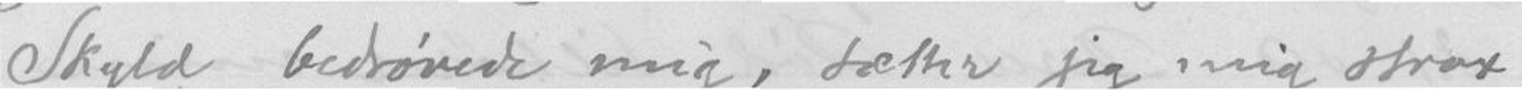Skyld bedrøvede mig, sætter jeg mig strax
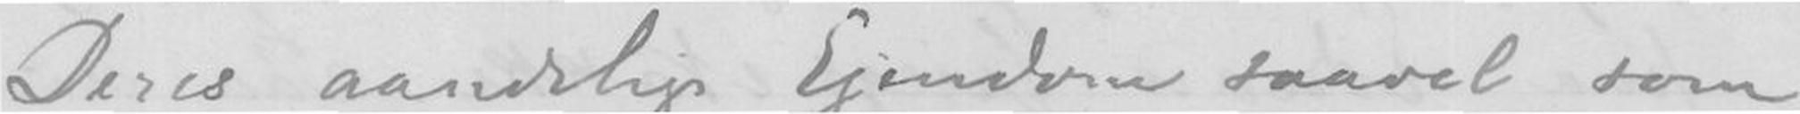Deres aandelige Ejendom saavel som
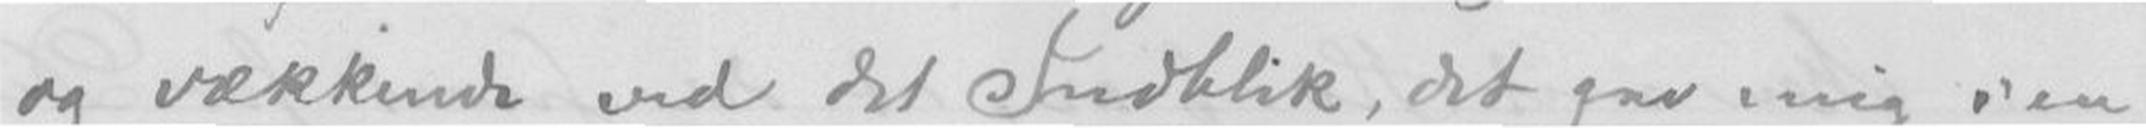og vækkende ved det Indblik, det gav mig i en
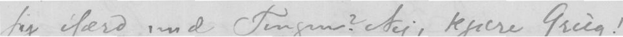sig ifærd med Tingen? Nej, kjære Grieg!
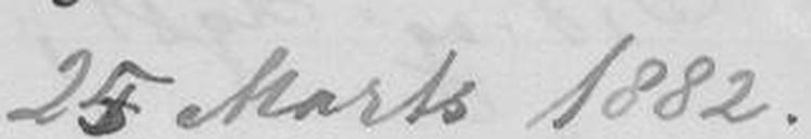25 Marts 1882.
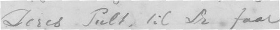Deres Pult, til De faar
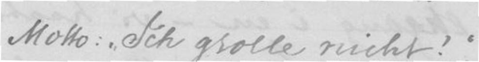Motto: Ich grolle nicht!
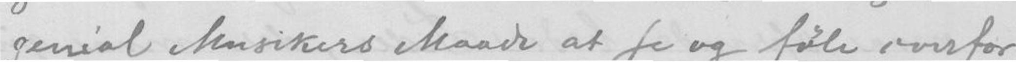genial Musikers Maade at se og føle overfor
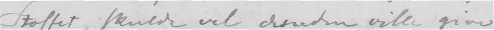Stoffet, skulde vel desuden ville give
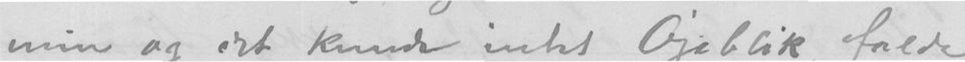min og det kunde intet Øjeblik falde
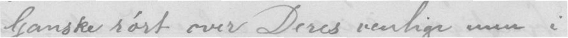Ganske rørt over Deres venlige men i
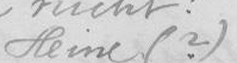Heine(?)
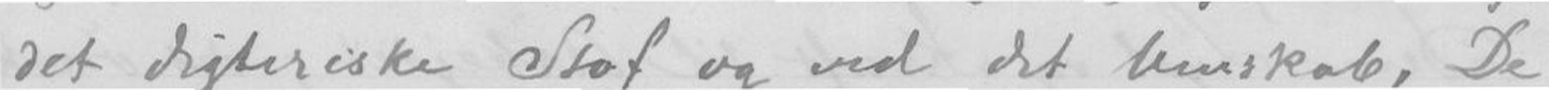det digteriske Stof og ved det venskab, De
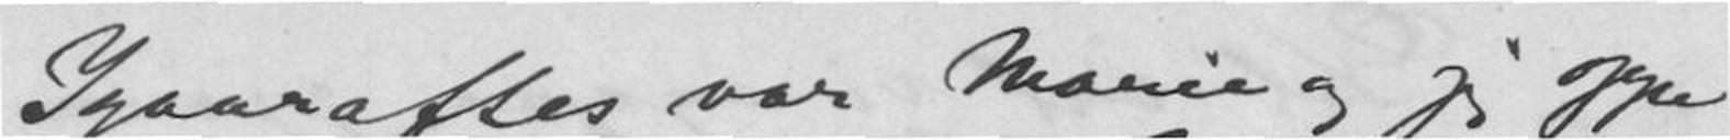Igaaraftes var Marie og jeg oppe
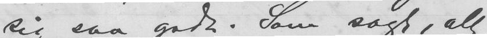sig saa godt. Som sagt, alt
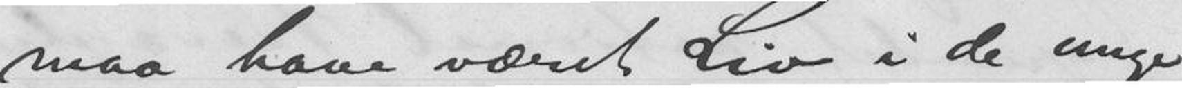maa have været Liv i de unge
